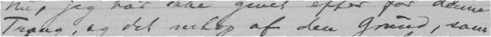Trang, og det netop af den Grund, som
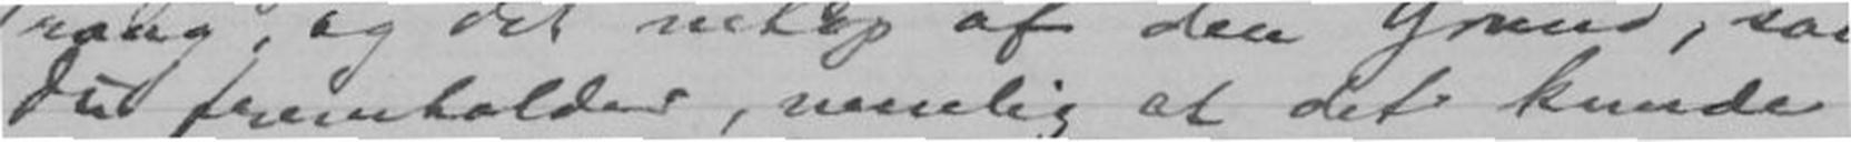Du fremholder, nemlig at det kunde
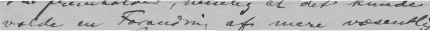volde en Forandring af mere væsentlig
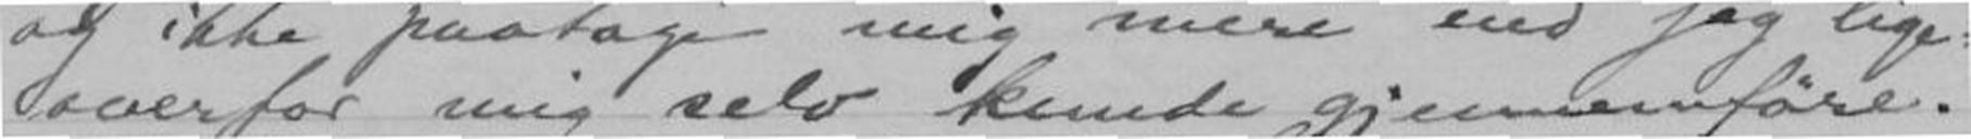overfor mig selv kunde gjennemføre.
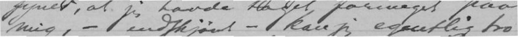mig, - endskjønt - kam jeg egentlig tro
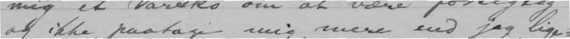og ikke paatage mig mere end jeg lige-
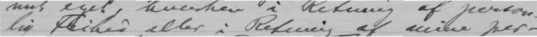lig Frihed eller i Retning af mine per-
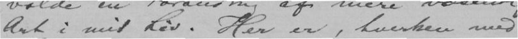Art i mit Liv. Her er, hverken med
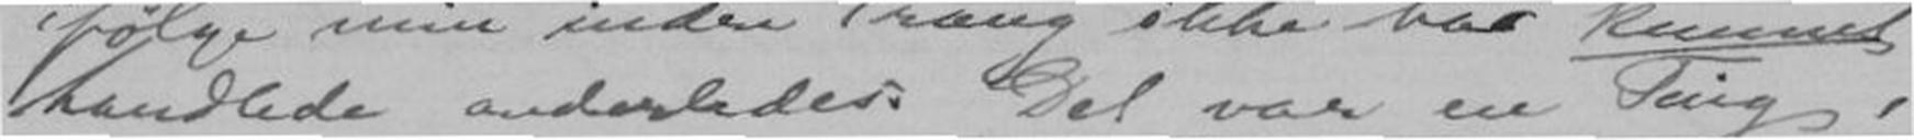handlede anderledes. Det var en Ting,
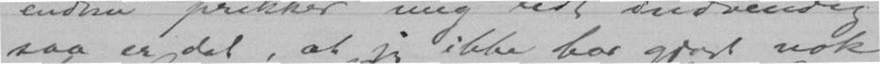saa er det, at jeg ikke har gjort nok
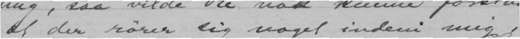at der rører sig noget indeni mig,
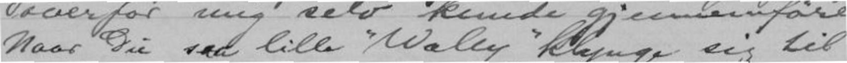Naar Du saa lille "Wally" klynge sig til
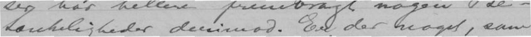tænkeligheder derimod. Er der noget, som
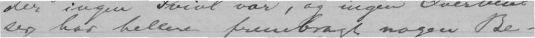ser har hellere frembragt nogen Be-
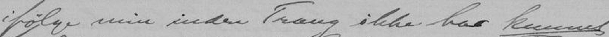ifølge min indre Trang ikke har kunnet
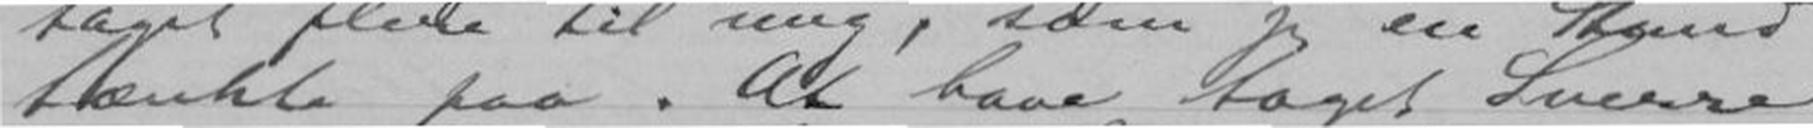tænkte paa. At have taget Sverre
